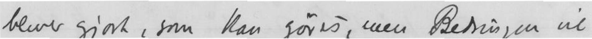bliver gjort, som kan gøres, men Bedringen vil
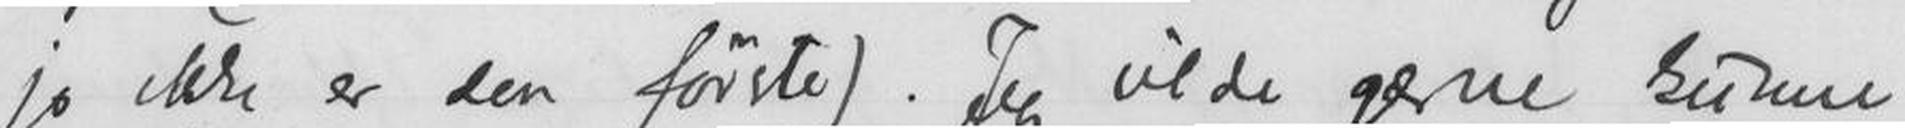jo ikke er den første). Jeg vilde gærne kunne
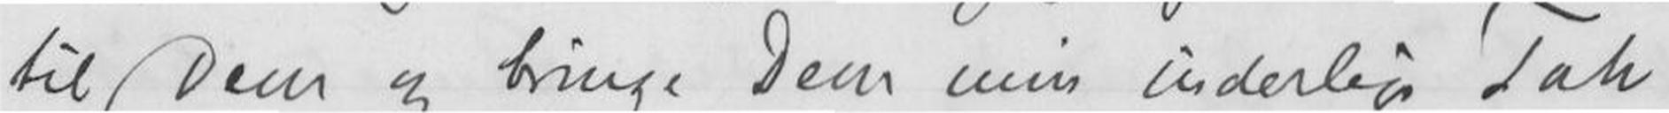til Dem og bringe Dem min inderlige Tak
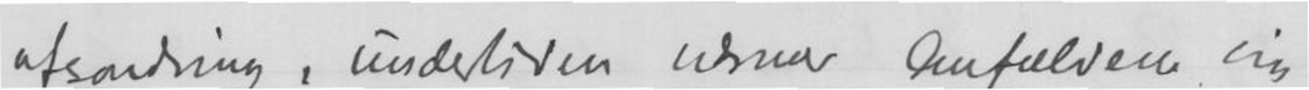afsondring, undertiden endnu Anfaldene om-
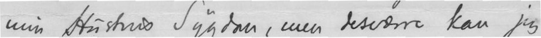min Hustrus Sygdom, men desværre kan jeg
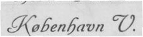København V.
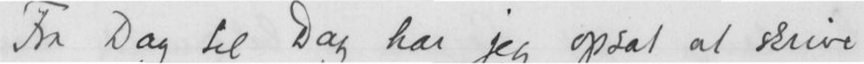Fra Dag til Dag har jeg opsat at skrive
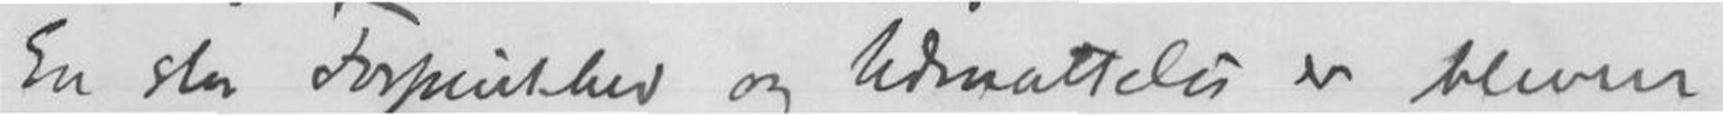En stor Forpinthed og Udmattelse er bleven
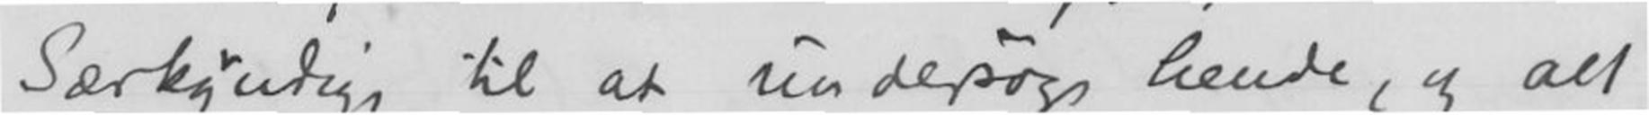Særkyndige til at undersøge hende, og alt
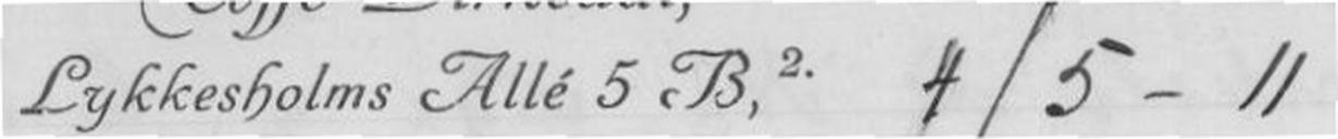Lykkesholms Allé 5B,2.4/5-11
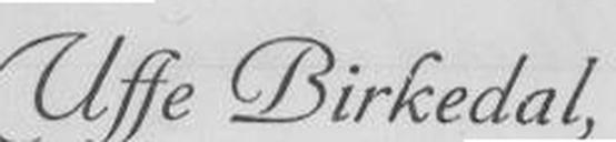Uffe Birkedal,
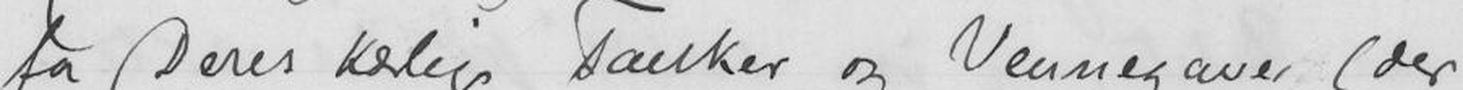for Deres kærlige Tanker og Vennegave (der
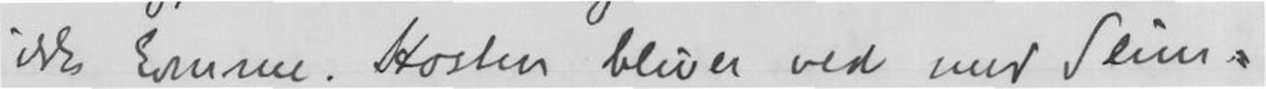ikke komme. Hosten bliver ved med Slim-
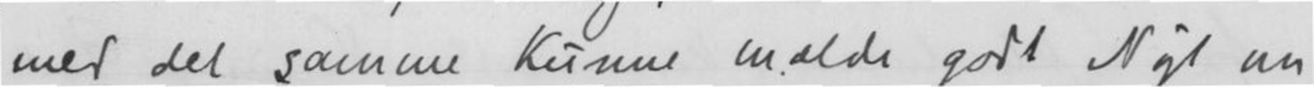med det samme kunne mælde godt Nyt om
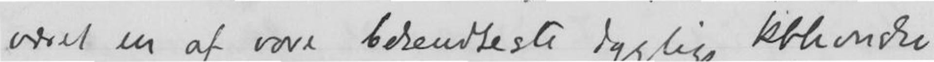udset en af vore bekendteste dygtige kbhvnske
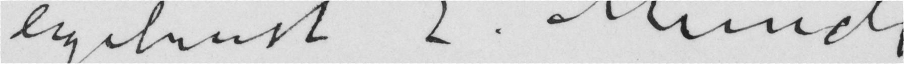ergebenst E. Munch
